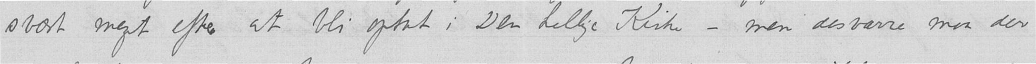svært meget efter at bli optat i Den hellige Kirke – men desværre maa der
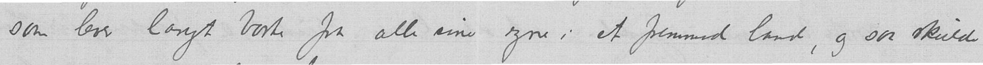som lever langt borte fra alle sine egne i et fremmed land, og saa skulde
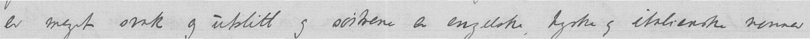er meget svak og utslitt og søstrene er engelske, tyske og italienske nonner
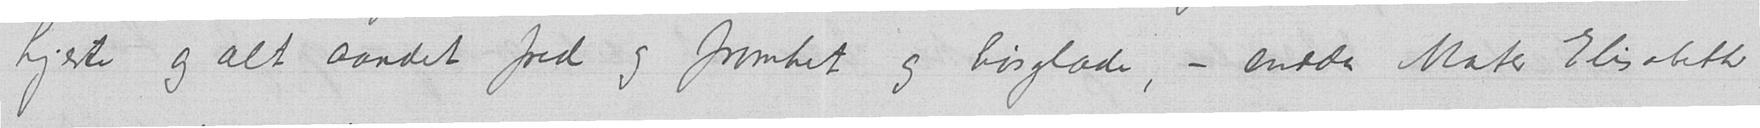hjerte og alt aandet fred og fromhet og livsglæde, - endda Mater Elisabeth
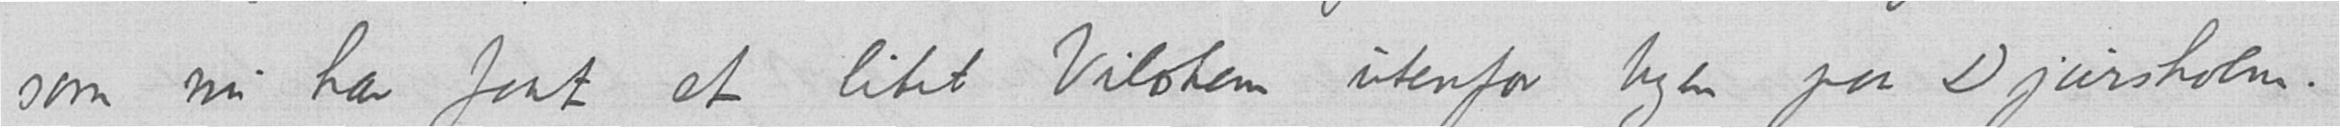som nu har faat et litet Vilohem utenfor byen paa Djursholm.
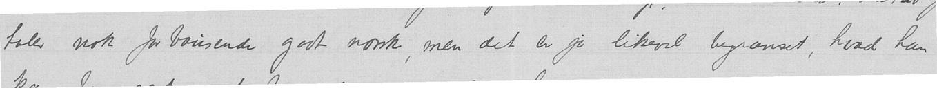taler nok forbausende godt norsk, men det er jo likevel begrænset, hvad han
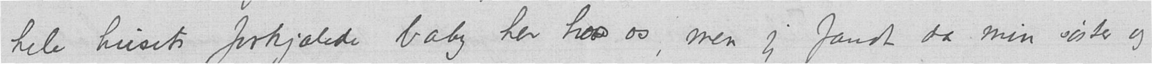hele husets forkjælede baby her hos os, men jeg fandt da min søster og
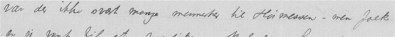var der ikke svært mange mennesker til Høimessen – men folk
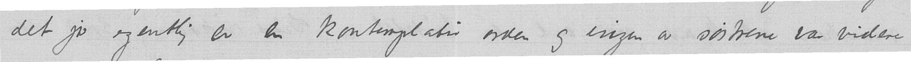det jo egentlig er en kontemplativ orden og ingen av søstrene var videre
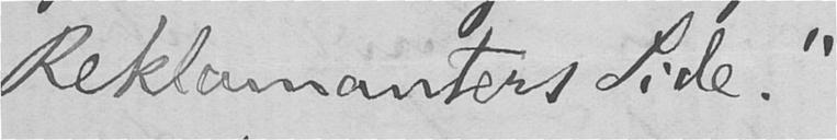Reklamanters Side."
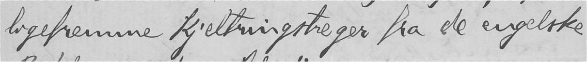ligefremme Kjeltringstreger fra de engelske
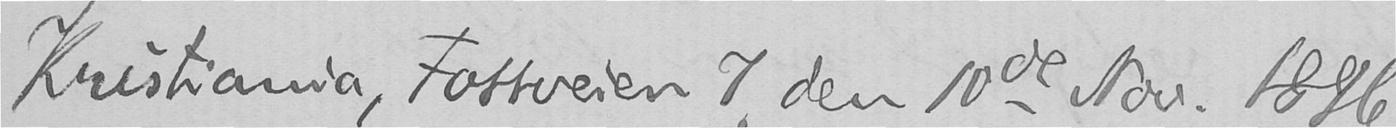Kristiania, Fossveien 7, den 10de Nov. 1896.
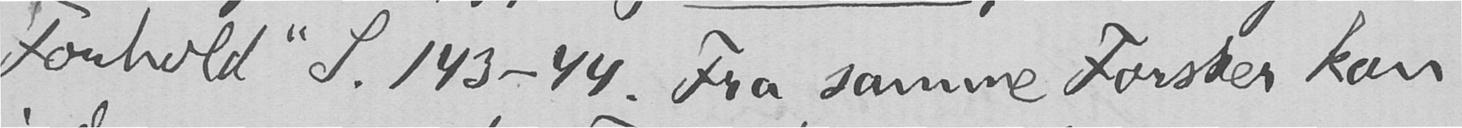forhold" S. 143-44. Fra samme Forsker kan
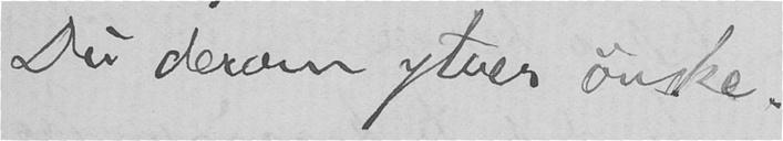Du derom ytrer ønske.
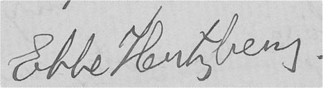Ebbe Hertzberg.
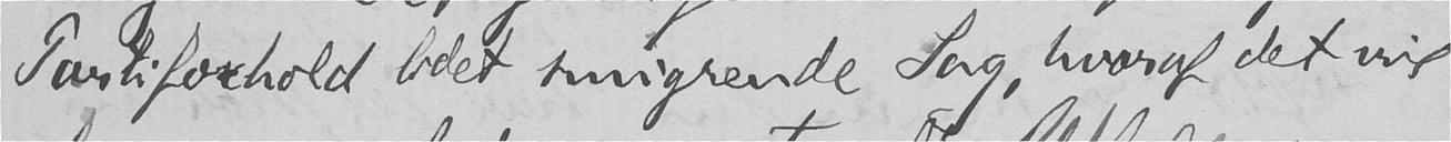Partiforhold lidet smigrende Sag, hvoraf det vil
